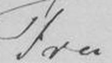Fra
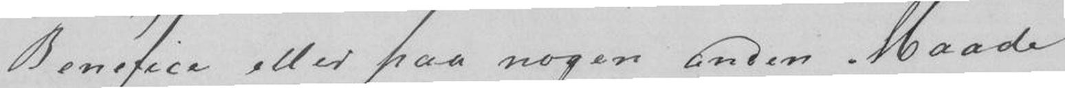Benefice eller paa nogen anden Maade
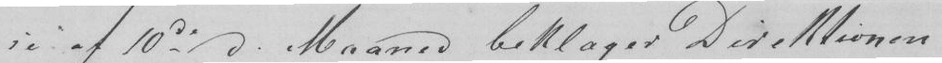se af 10de d. Maaned beklager Direktionen
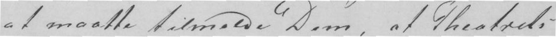at maatte tilmelde Dem, at Theatrets
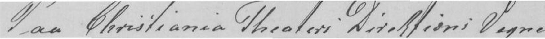Paa Christiania Theaters Direktions Vegne
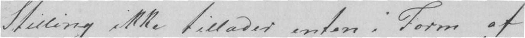Stilling ikke tillader enten i Form af
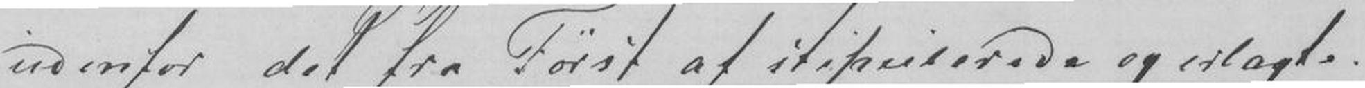udenfor det fra Først af stipulerede og erlagte.
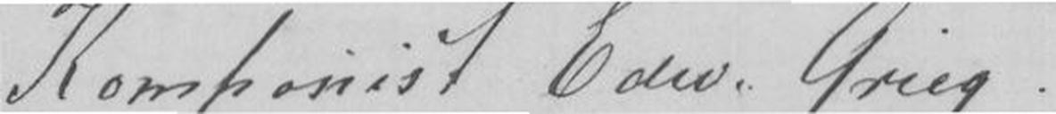Komponist Edv. Grieg.
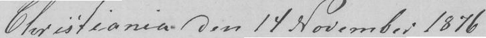Christiania den 14. November 1876
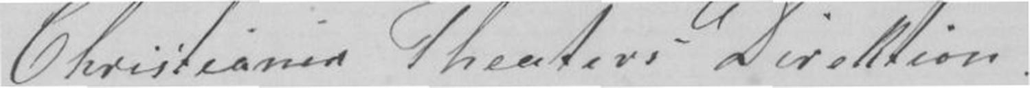Christiania Theaters Direktion.
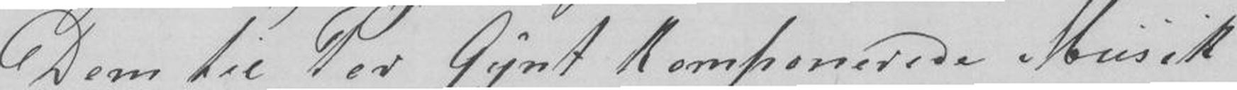Dem til Per Gynt komponerede Musik
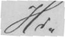Hr.
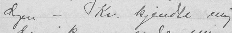dagen - Kr. kjendte mig
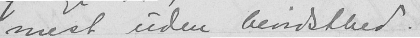mest uden bevidsthed.
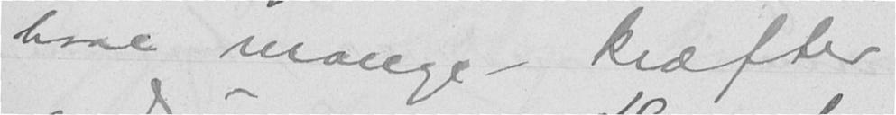have mange kræfter
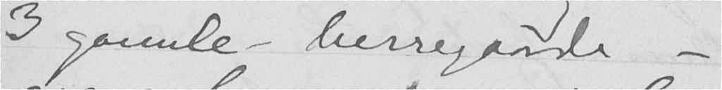3 gamle herregaarde -
